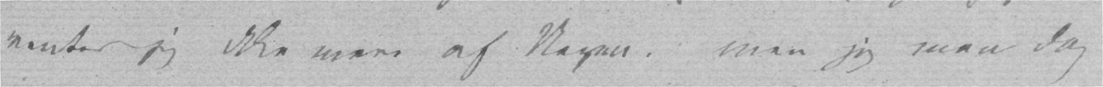venter jeg ikke mere af Nogen, men jeg maa dog
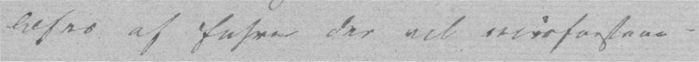læses af Enhver der vil misforstaae.
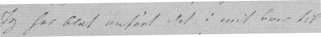Jeg har blot anført det i mit Brev til
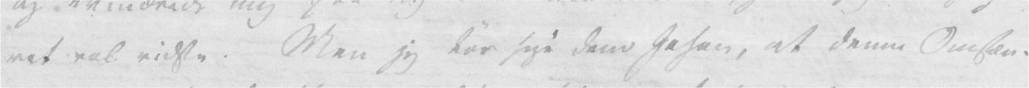ret vel vidste. Men jeg tør sige Dem Johan, at denne Omstæn-
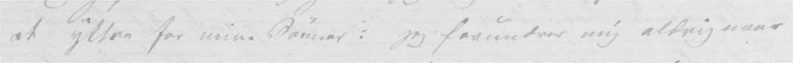at yttre for mine Sønner: jeg forundrer mig aldrig naar
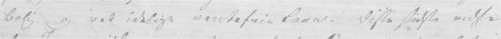Bolig og ved idelige rentefrie Laan. Disse sidste vidste
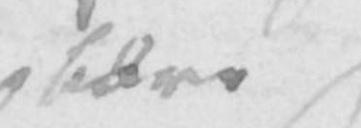bære
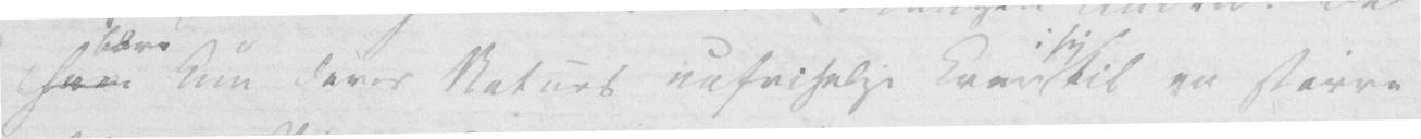have kun deres Naturs uafviselige Krav i sig til en større
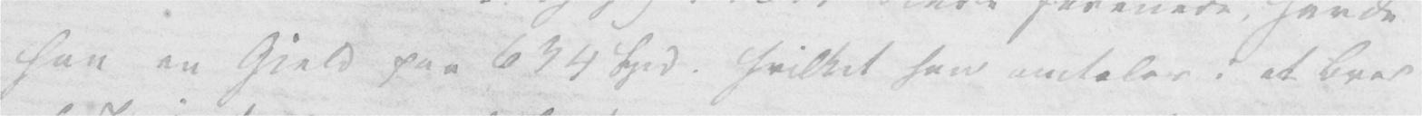han en Gjeld paa 634 Spd. hvilket han omtaler i et Brev
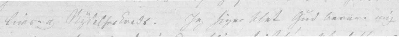Livs- og Nydelseskreds. Jeg siger blot Gud bevare mig
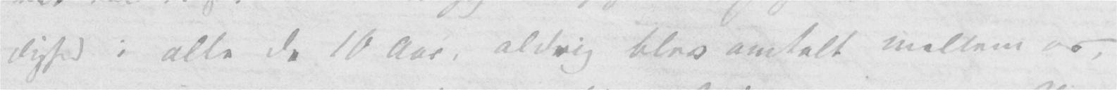dighed i alle de 10 Aar, aldrig blev omtalt mellem os,
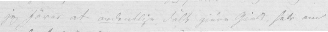jeg hører at ordentlige folk gjøre Gjeld, selv om
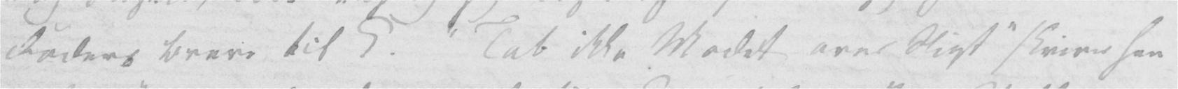Faders Breve til C. «Tab ikke Modet over Sligt» skriver han
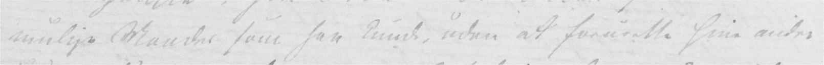mulige Maader som han kunde, uden at forurette sine andre
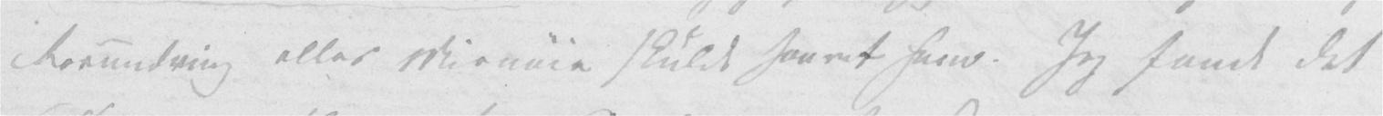Forundring eller Misnøie skulde saaret ham. Jeg fandt det
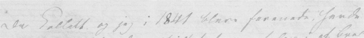Da Collett og jeg i 1841 bleve forenede, havde
